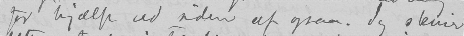for hjælp ved siden af ogsaa. Jeg skriver
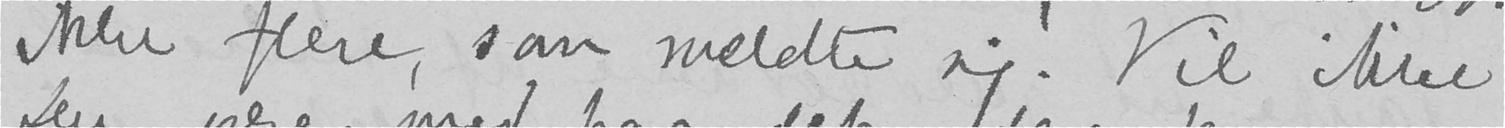ikke flere, som meldte sig. Vil ikke
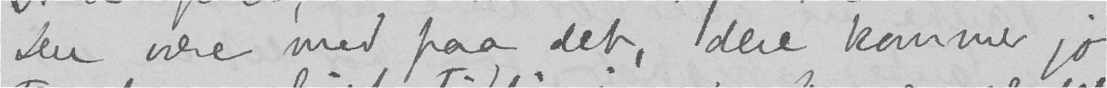Du være med paa det, dere kommer jo
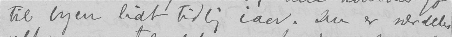til byen lidt tidlig iaar. Du er særdeles
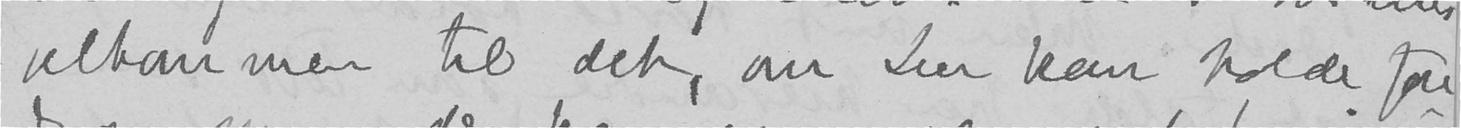velkommen til det, om Du kan holde fore-
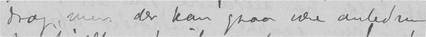drag, men der kan ogsaa være anledning
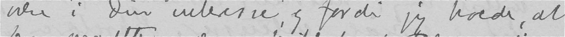være i Din interesse, og fordi jeg troede, at
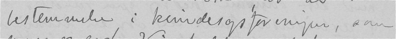bestemmelse i kvindesagsforeningen, som
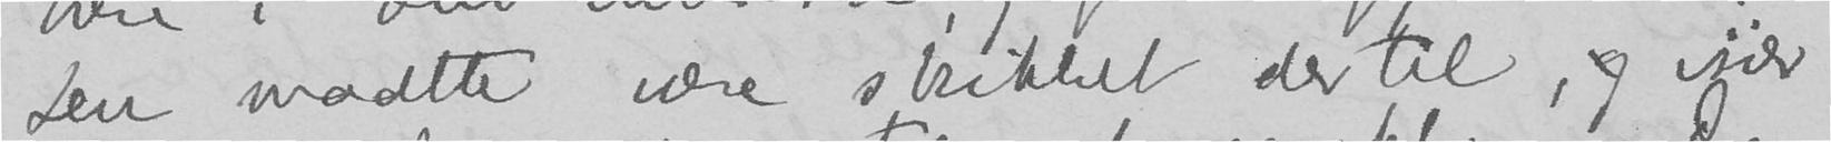Du maatte være skikket dertil, og især
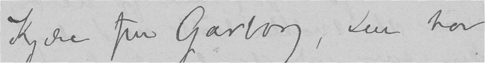Kjære fru Garborg, Du har
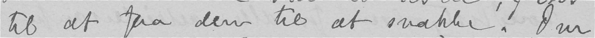til at faa dem til at snakke. Om
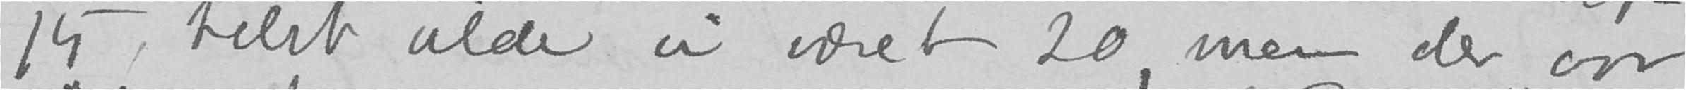15, helst vilde vi været 20, men der var
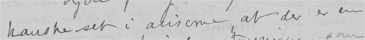kanske set i aviserne, at der er en
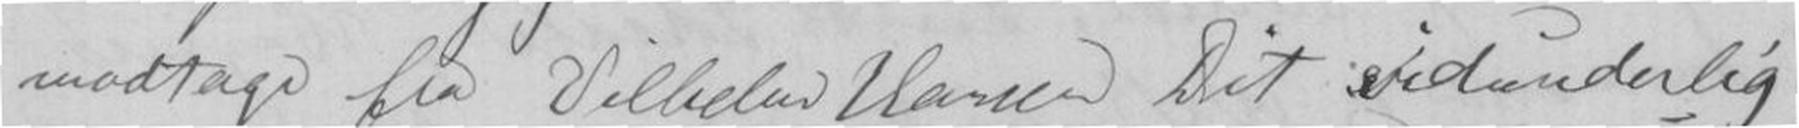modtage fra Vilhelm Hansen Dit vidunderlig
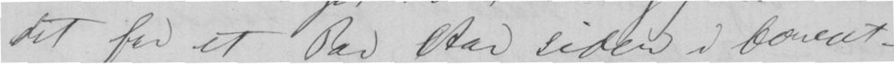det for et Par Aar siden i Concert-
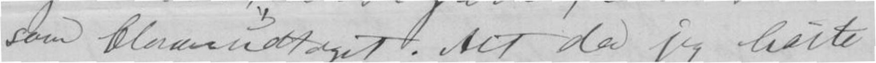som Claverudtaget. Alt da jeg hørte
